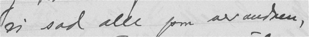vi sad alle paa verandaen.
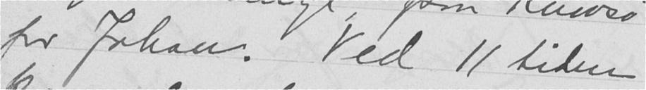for Johan. Ved 11 tiden
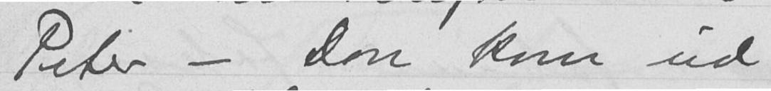Peter - Don kom ud
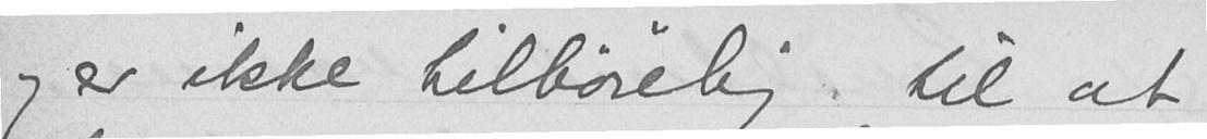og er ikke tilbøielig til at
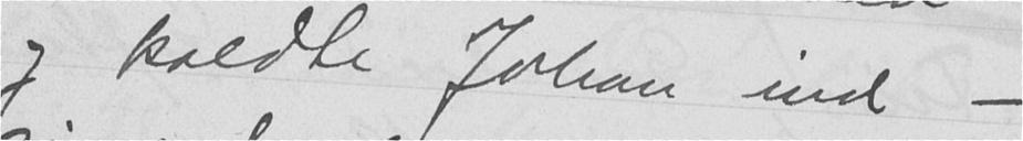og kaldte Johan ind -
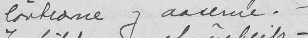løvtrærne og aaserne. -
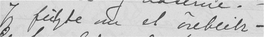Jeg fulgte om et øieblik -
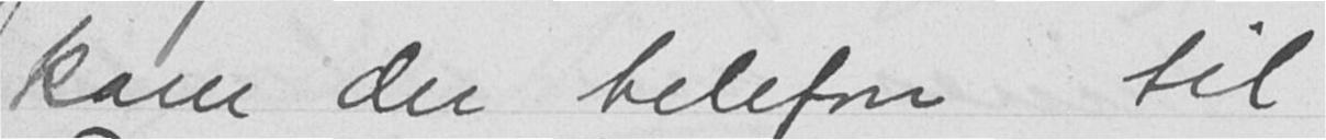kom der telefon til
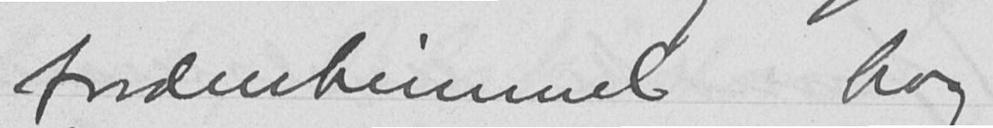tordenhimmel bag
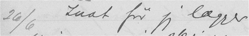26/6 Inat før jeg lægger
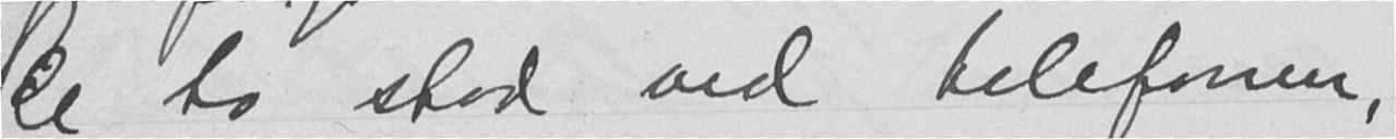de to stod ved telefonen,
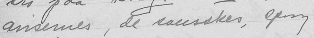avisernes, de svenskes, sprog
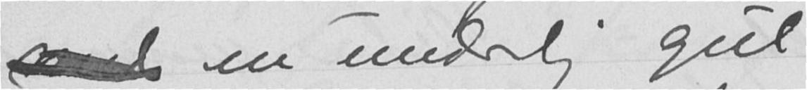ved en underlig gul
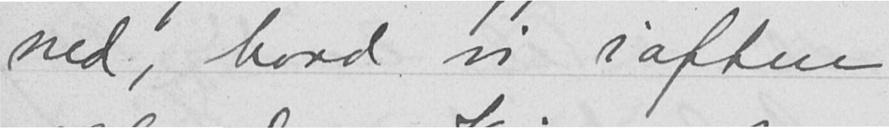ned, hvad vi iaften
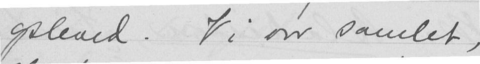opleved. Vi var samlet,
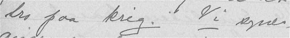tro paa krig. Vi synes
